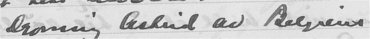Dronning Astrid av Belgiens
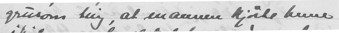grusom ting, at mannen kjørte henne
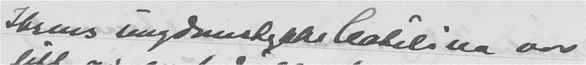Ibsens ungdomstykke Catalina var
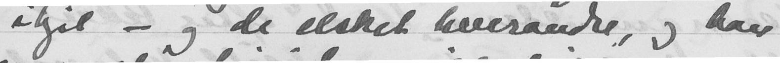ihjel - og de elsket hverandre, og han
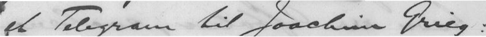et Telegram til Joachim Grieg:
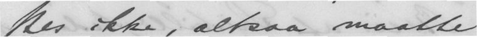stes ikke, altsaa maatte
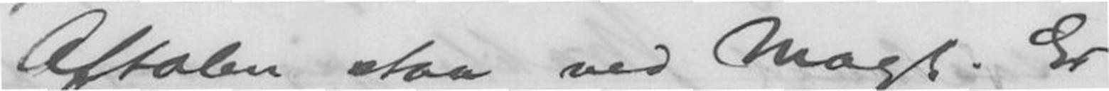Aftalen staa ved Magt. Er
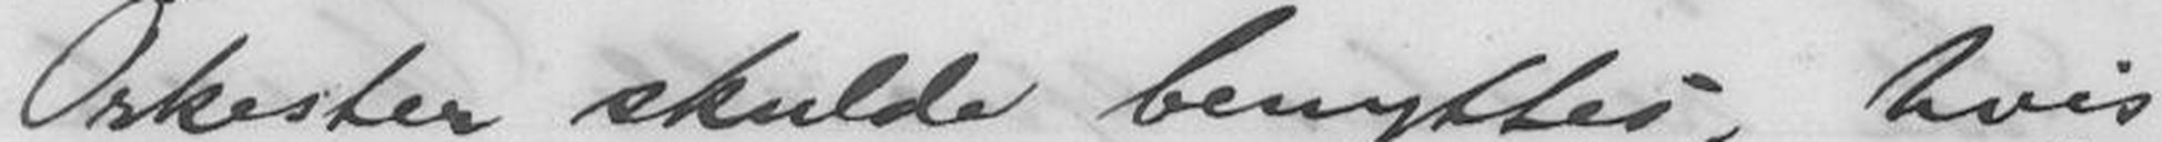Orkester skulde benyttes, hvis
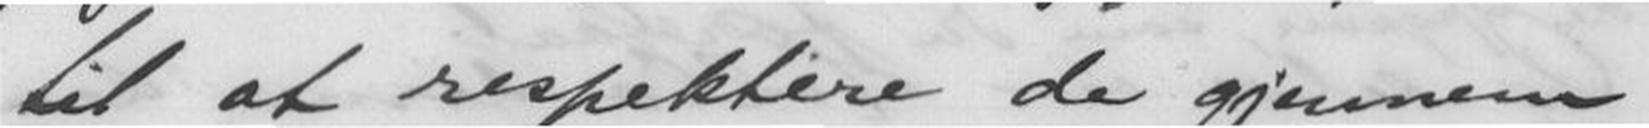til at respektere de gjennem
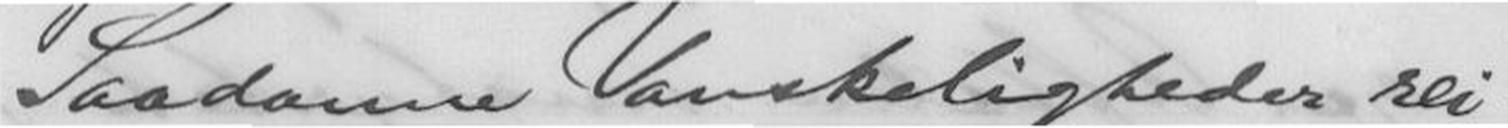Saadanne Vanskeligheder rei-
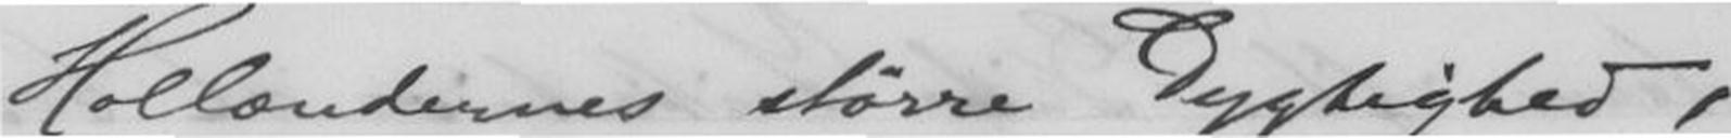Hollændernes større Dygtighed,
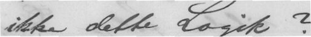ikke dette Logik?
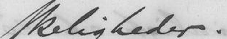skeligheder.
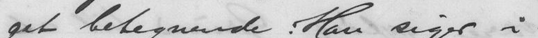get betegnende. Han siger i
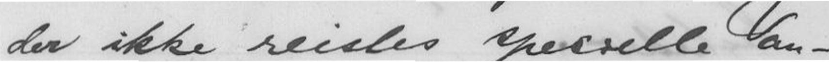der ikke reistes spesielle Van-
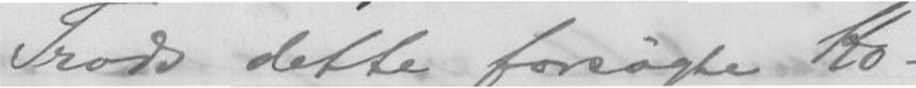Trods dette forsøgte Ko-
